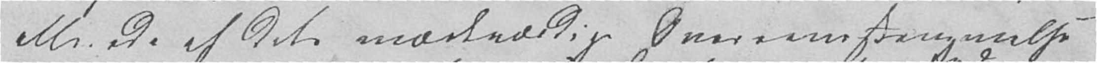allerede af dets mærkværdige Overeenstemmelse
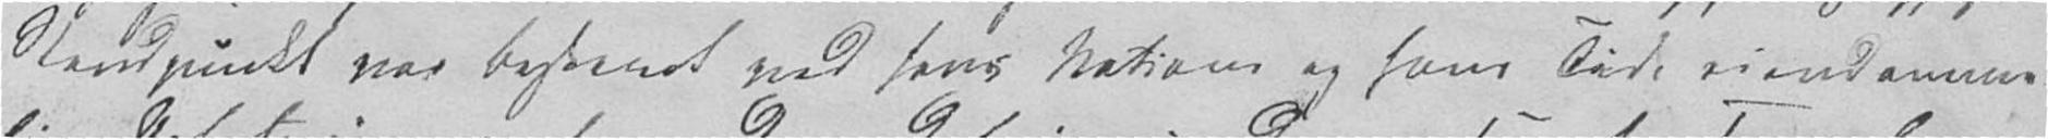Standpunkt var bestemt ved hans Nation og hans Tids eiendomme-
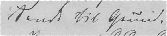Sandt til Grund.
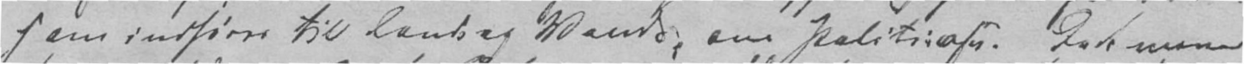som indføres til Lands og Vands, om Politi osv. Det mener
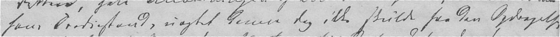hans Trediestand, uagtet denne dog ikke skulde faa den Opdragelse,
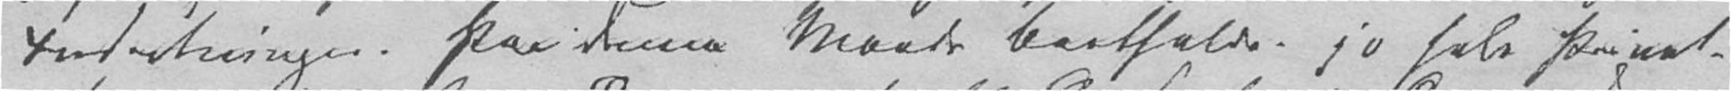Indretninger. Paa denne Maade bortfalder jo hele Privat-
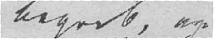begreb, og
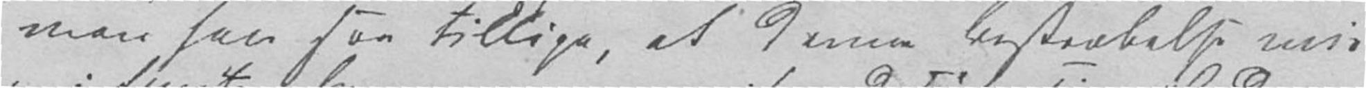men han saa tillige at denne Bestræbelse mis-
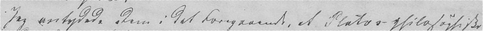Jeg antydede Dem i det Foregaaende, at Platos philosophiske
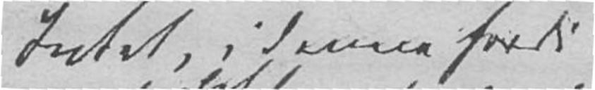Intet, i denne fordi
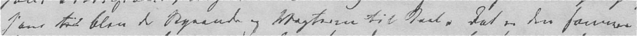som til blev de Styrende og Vogterne til Deel. Det er den samme
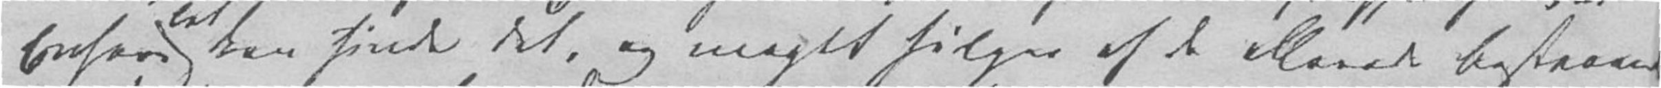Enhver kan finde det, og meget følger af de allerede bestaaende
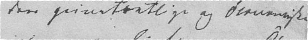den privatretlige og øconomiske
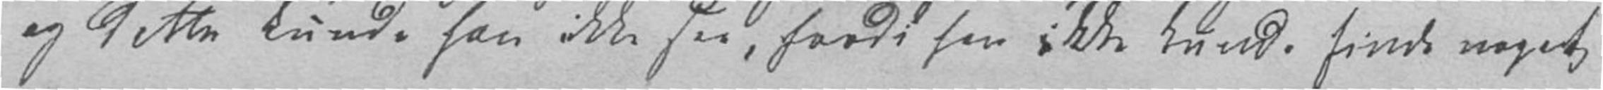og dette kunde han ikke see, fordi han ikke kunde finde noget,
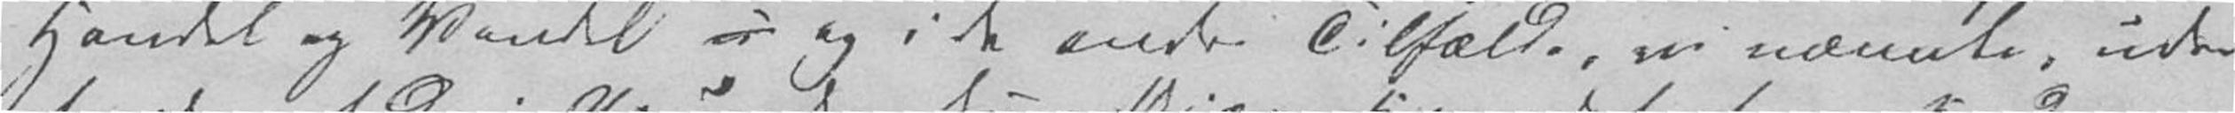Handel og Vandel og i de andre Tilfælde, vi nævnte, uden
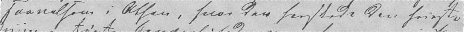saavelsom i Athen, hvor der herskede den frieste
